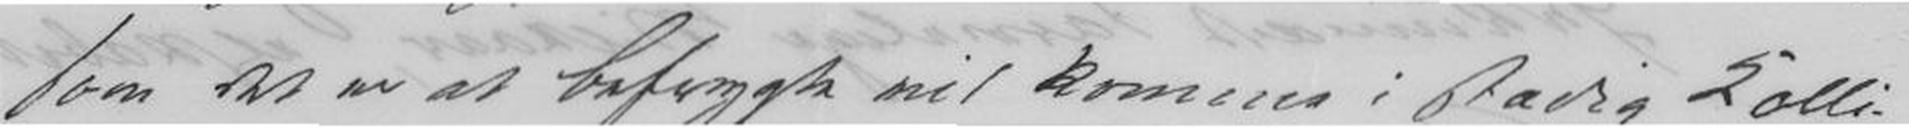som det er at befrygte vil komme i stadig Kolli-
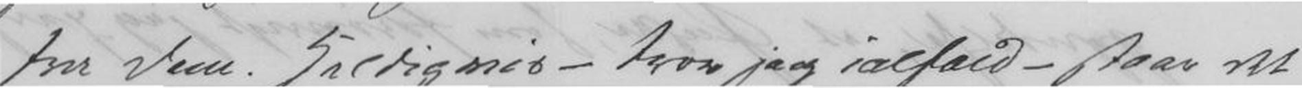fra dem. Heldigvis - tror jeg ialfald - staar det
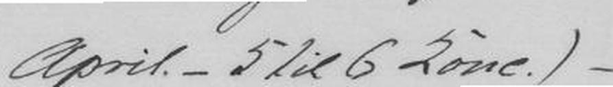April. - 5 til 5 Konc.)-
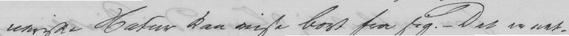neriske Natur kan ønske bort fra sig. - Det er net-
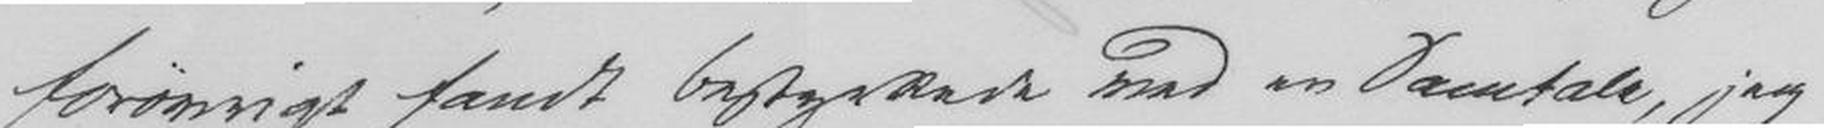forøvrigt fandt bestyrkede med en Samtale, jeg
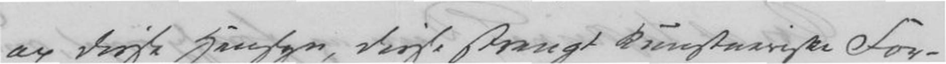op disse Hensyn, disse strengt kunstneriske For-
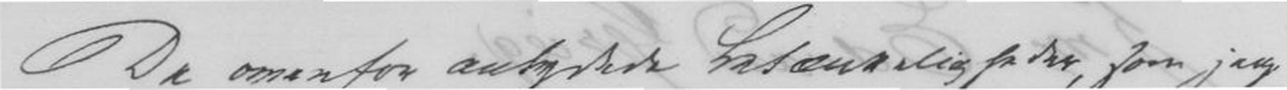De overfor antydede Betænkeligheder, som jeg
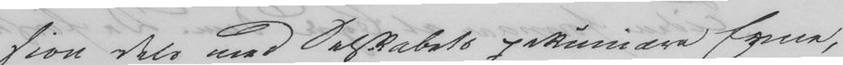sion dels med Selskabets pekuniære Evne,
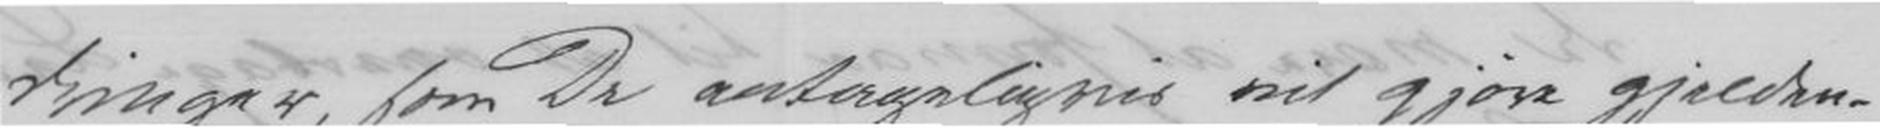dringer, som De antageligvis vil gjøre gjelden-
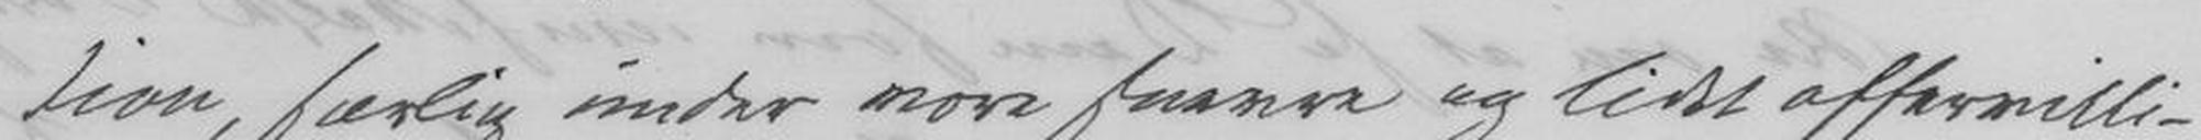tion, særlig under vore snarere og lidt offervilli-
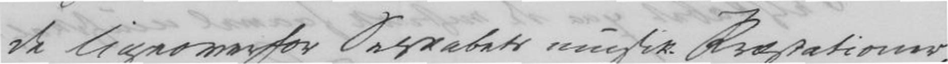de ligeoverfor Selskabets musik. Præstationer,
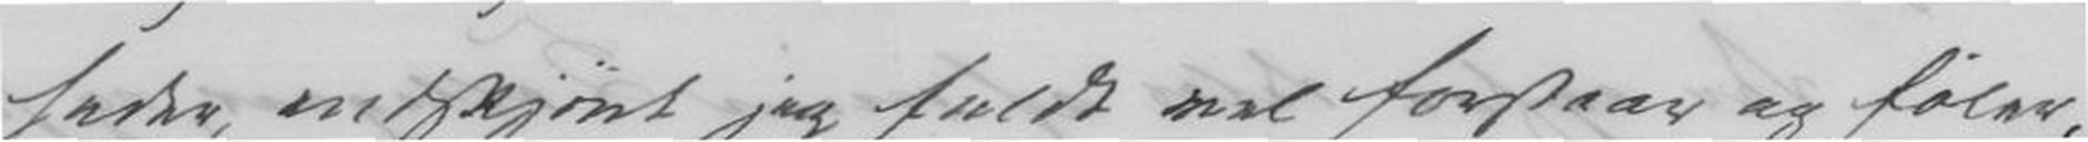heder, endskjønt jeg fuldt vel forstaar og føler,
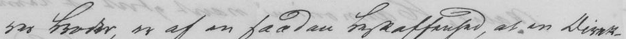res Broder, er af en saadan Beskaffenhed, at en Direk-
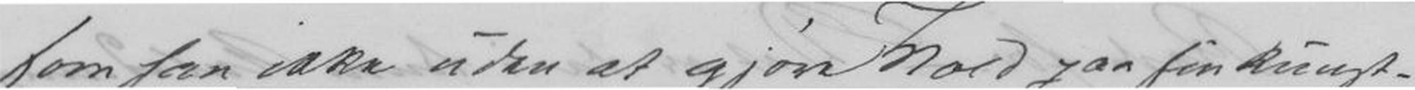som han ikke uden at gjøre Vold paa sin kunst-
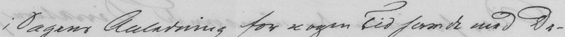i Sagens Anledning for nogen Tid havde med De-
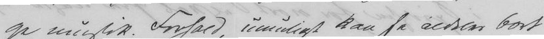ge musik Forhold, umuligt kan se aldeles bort
